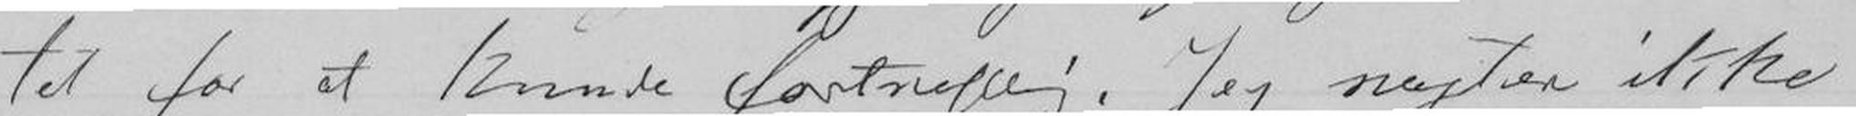til for at kunde fortreflig. Jeg nægter ikke
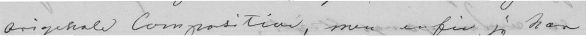originale Composition, men er fri jeg har
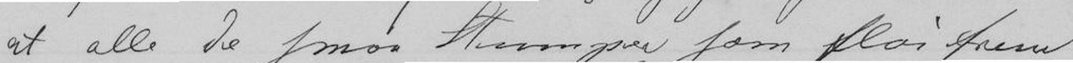at alle de smaa Stumper som fløi frem
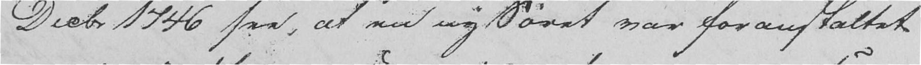Decbr 1746 see, at en ny Søret var foranstaltet
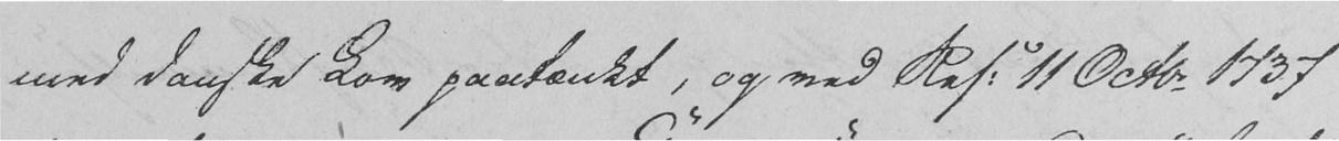med danske Lov paatænkt, og med Res. 11 Octbr 1737
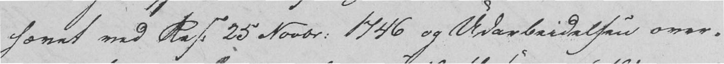hævet ved Res. 25 Novbr. 1746 og Udarbeidelsen over-
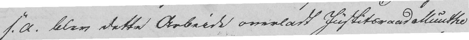s. A. blev dette Arbeide overladt Justitsraad Munthe
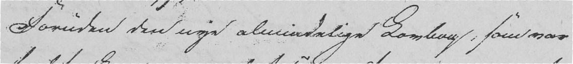Foruden den nye almindelige Lovbog, som var
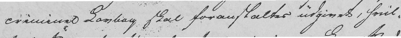criminel Lovbog skal foranstaltes udgivet, hvil-
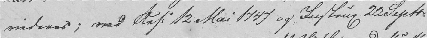videres; med Res. 12 Mai 1747 og Instrux 22 Septr
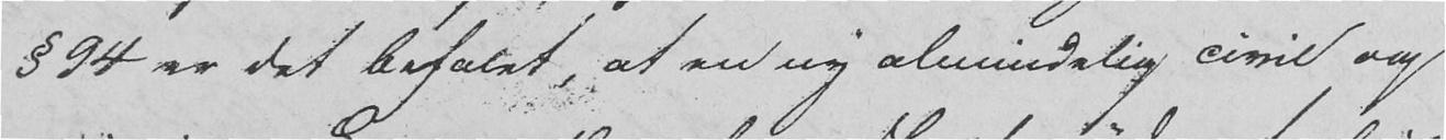§ 94 er det befalet, at en ny almindelig civil og
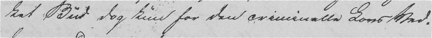ket Bud dog kun for den criminelle Lovs Ved-
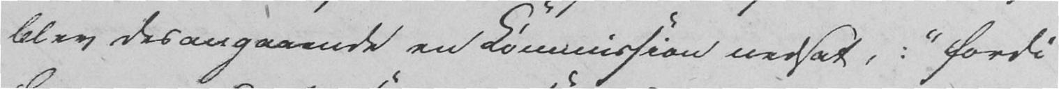blev desangaaende en Kommission nedsat, : "fordi
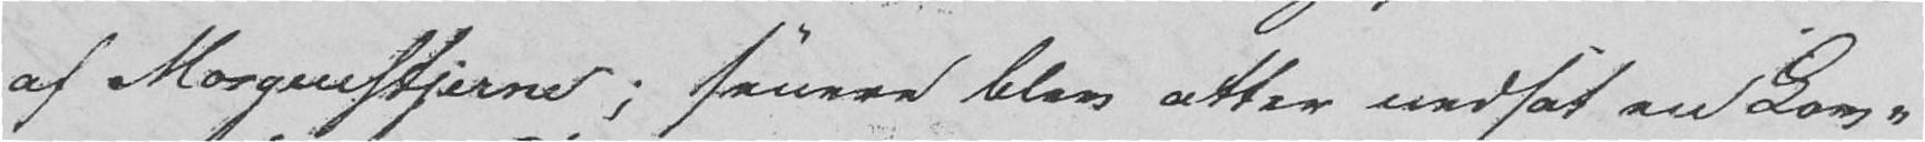af Morgenstjerne; senere blev atter nedsat en Lov-
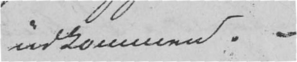udkommen. -
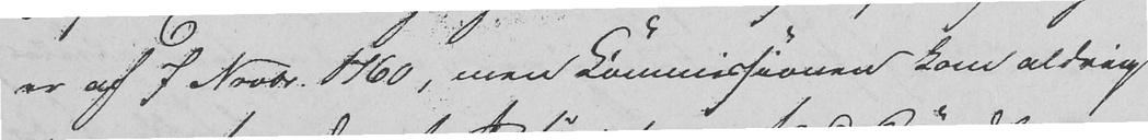er af 7 Novbr. 1760, men Kommissionen kom aldrig
